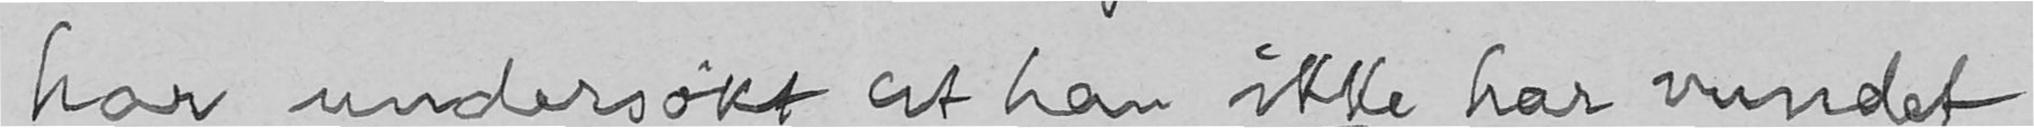har undersøkt at han ikke har vundet
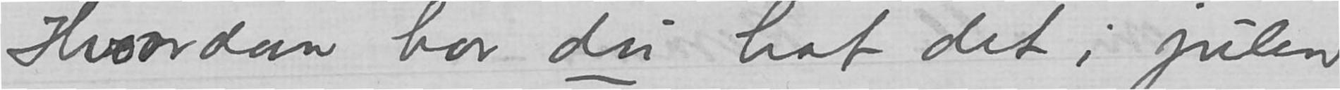Hvordan har du hat det i julen
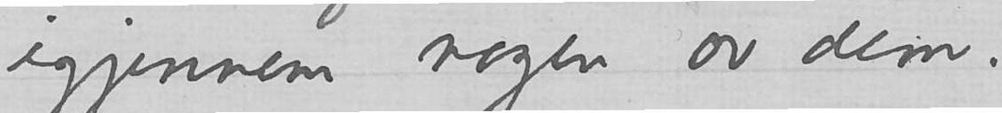igjennem nogen av dem.
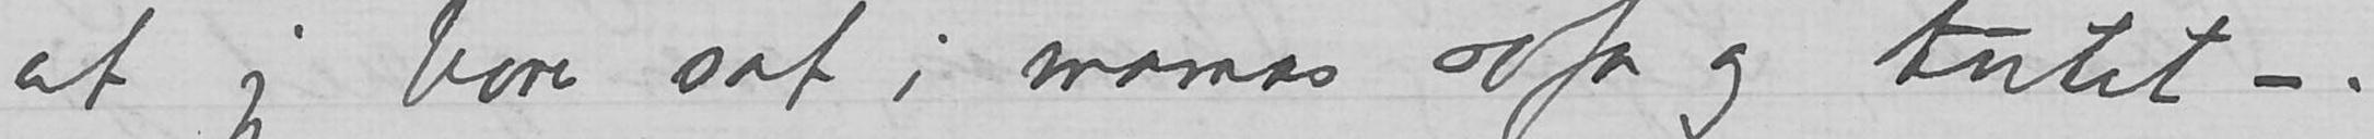at jeg bare sat i mamas sofa og tutet -.
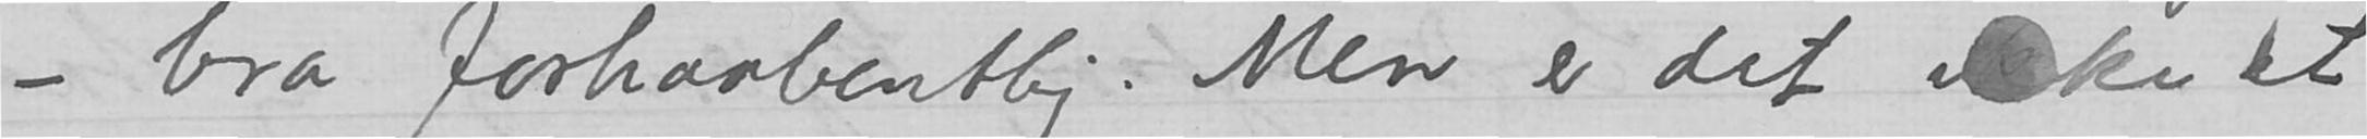– bra forhaabentlig. Men er det ikke et
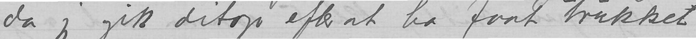da jeg gik ditop efter at ha faat trukket
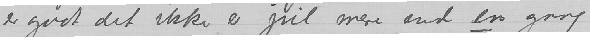er godt det ikke er jul mere end en gang
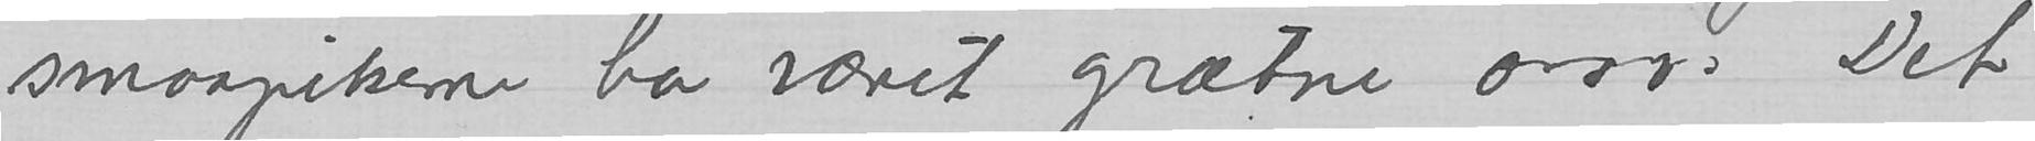smaapikerne har været grætne osv. Det
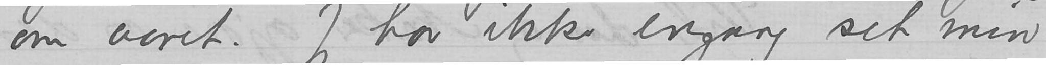om aaret. Jeg har ikke engang set min
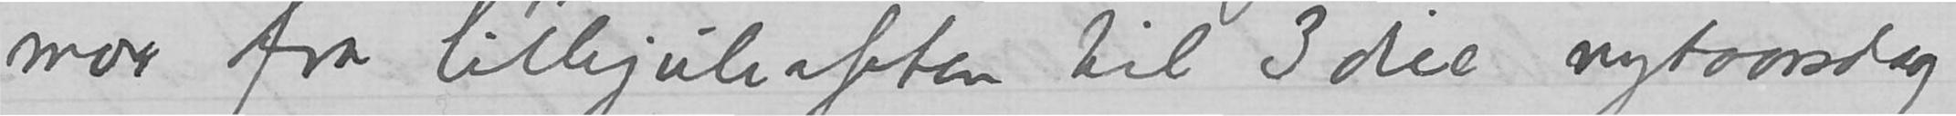mor fra lillejuleaften til 3die nytaarsdag
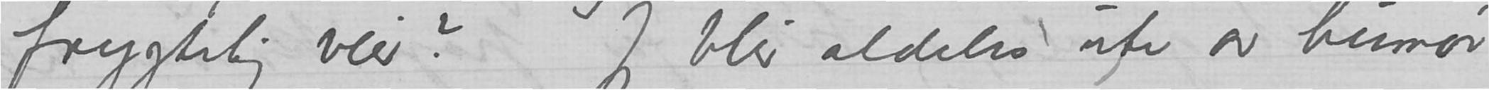frygtelig veir? Jeg blir aldeles ute av humør
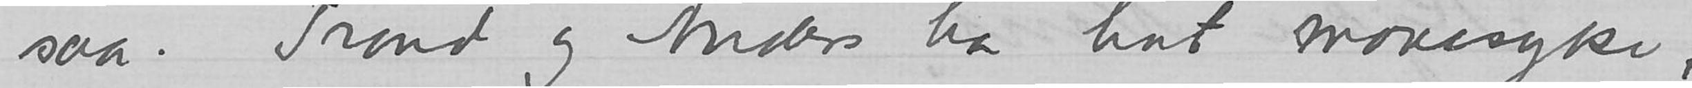saa. Trond og Anders har hat mavesyke,
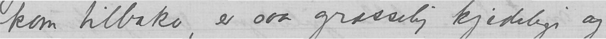kom tilbake, er saa græsselig kjedelige og
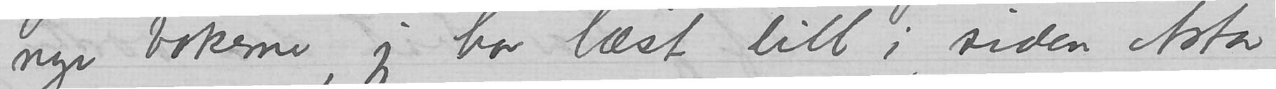nye bøkerne, jeg har læst litt i siden Asta
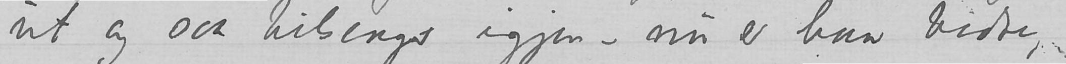ut og saa tilsengs igjen – nu er han bedre,
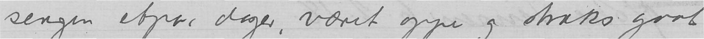sengen etpar dager, været oppe og straks gaat
